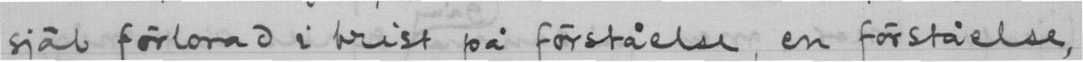själ förlorad i brist på förståelse, en förståelse,
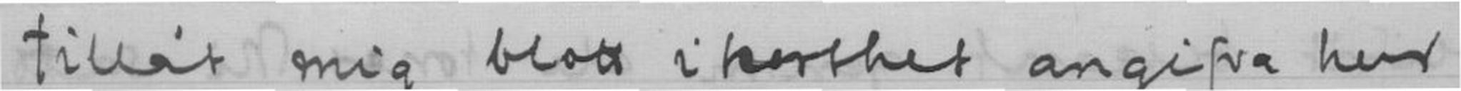Tillåt mig blott ikorthet angifva hur
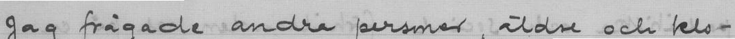Jag frågade andre personer, äldre och klo-
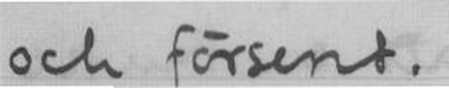och försent.
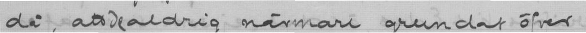då, att de aldrig närmare grundat öfver
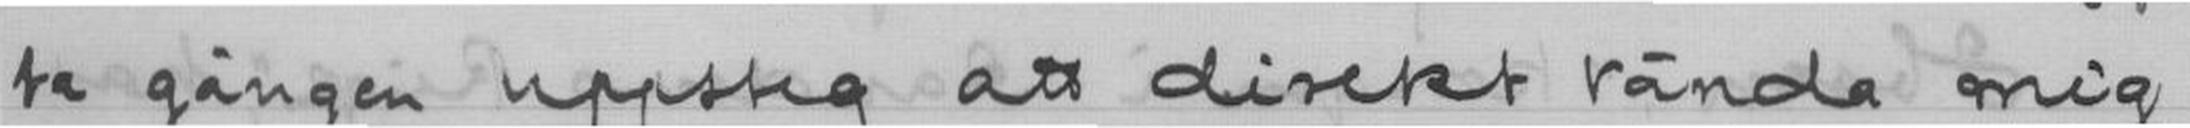ta gången uppsteg att direkt vända mig
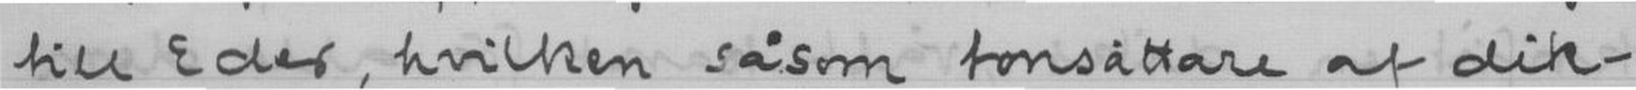till Eder, hvilken såsom tonsättare af dik-
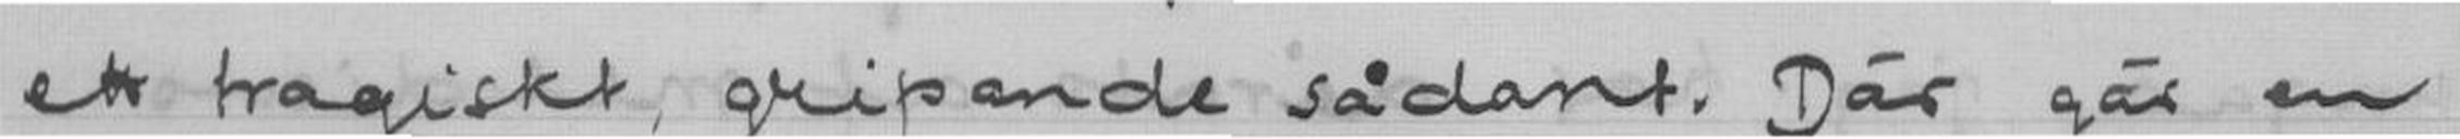ett tragiskt, gripande sådant. Där gär en
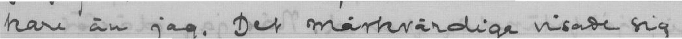kare än jag. Det märkvärdiga visade sig
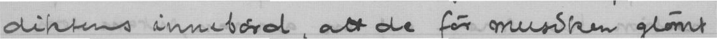diktens innebörd, att de för musiken glömt
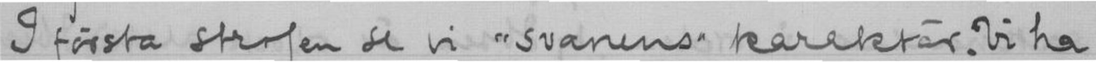I första strofen se vi "svanens" karektär. Vi ha
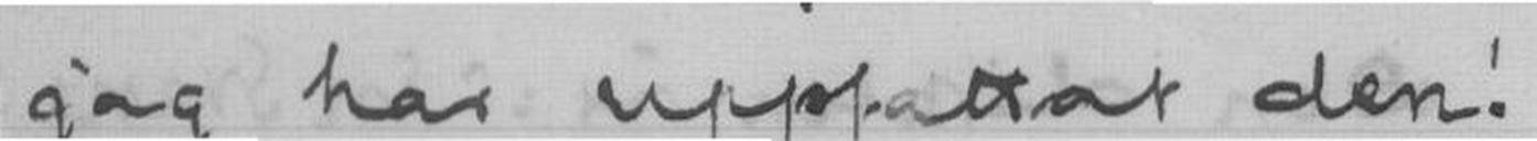jag har uppfattat den!
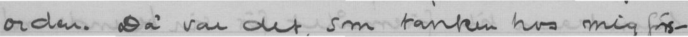orden. Då var det, som tanken hos mig förs-
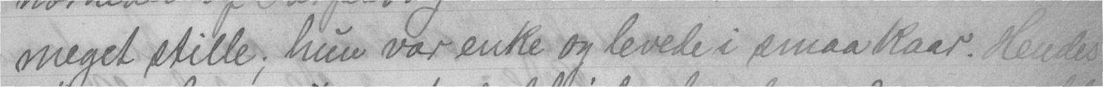meget stille, hun var enke og levede i smaa kaar. Hendes
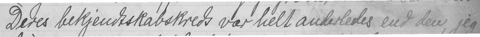Deres bekjendtskabskreds var helt anderledes end den, jeg
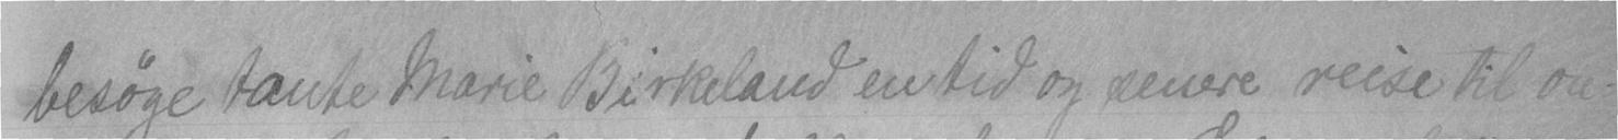besøge tante Marie Birkeland en tid og senere reise til on-
